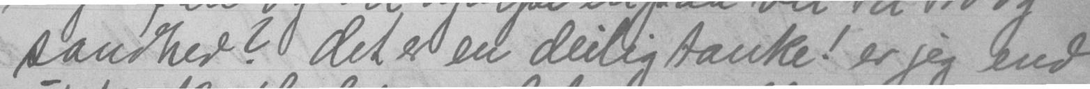sandhed? det er en deilig tanke! er jeg end
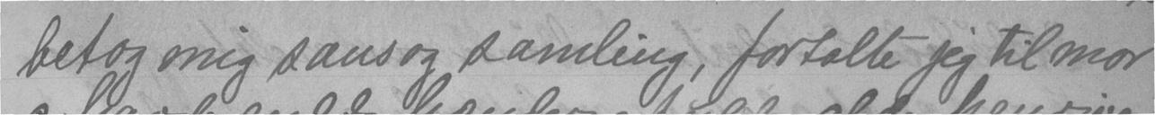betog mig sans og samling, fortalte jeg til mor
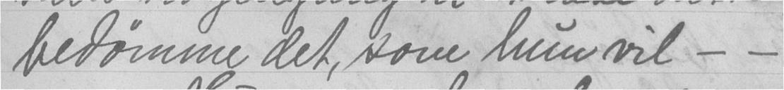bedømme det, som hun vil - -
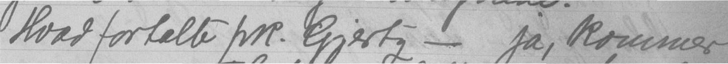Hvad fortalte frk. Gjertz - ja, kommer
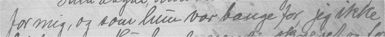for mig, og som hun var bange for jeg ikke
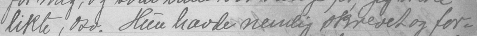likte, osv. Hun havde nemlig skrevet og for-
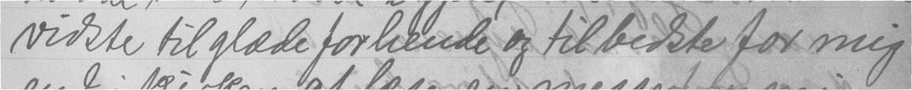vidste til glæde forhende og til bedste for mig
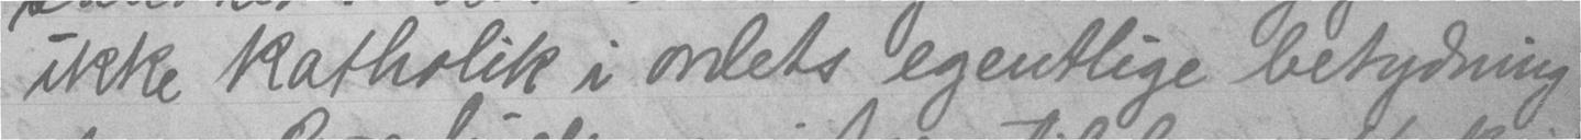ikke katholik i ordets egentlige betydning
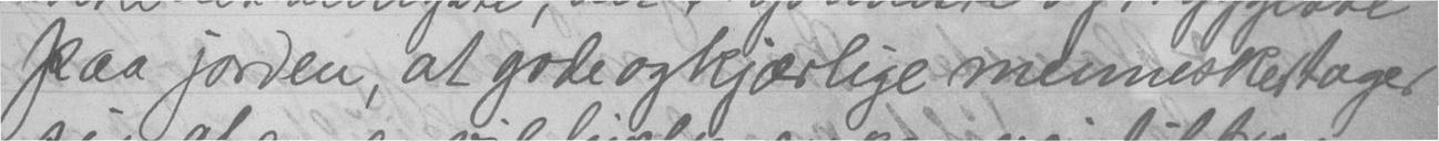paa jorden, at gode og kjærlige mennesker tager
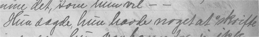Hun sagde, hun havde noget at "skrifte"
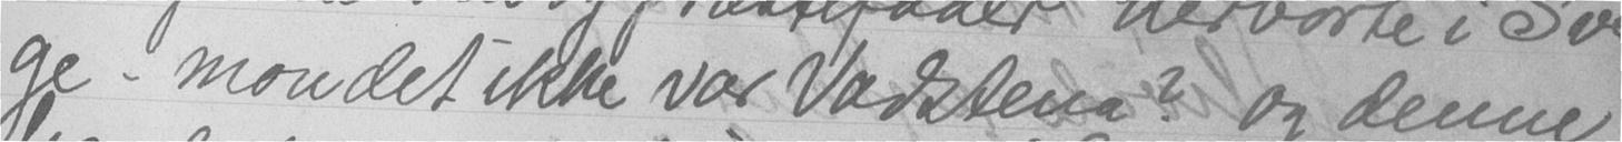ge - mon det ikke var Vadstena? og denne
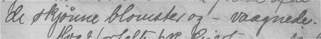de skjønne blomster og - vaagnede.
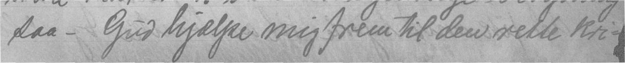saa - Gud hjælpe mig frem til den rette kri-
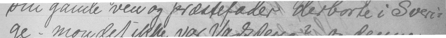sin gamle ven og præstefader, derborte i Sveri-
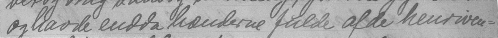og havde endda hænderne fulde af de henriven-
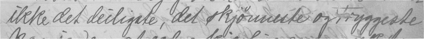ikke det deiligste, det skjønneste og tryggeste
